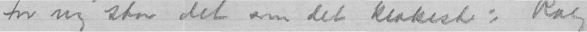For mig staar det som det klokeste: Ragt
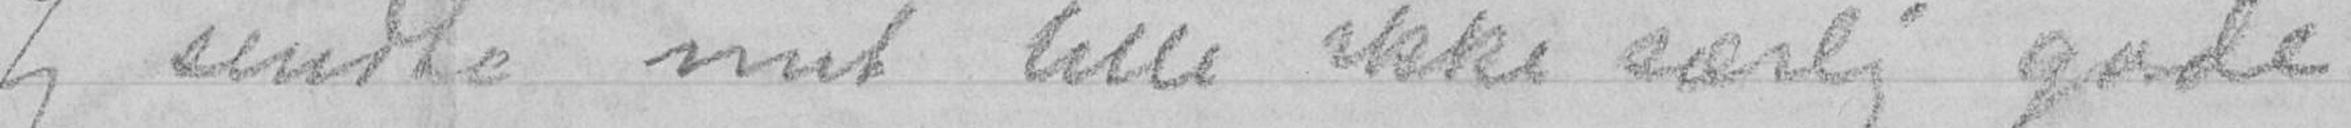Jeg sendte mit lille ikke særlig gode
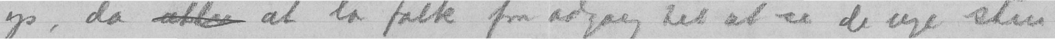op, da  at la folk faa adgang til at se de nye sten
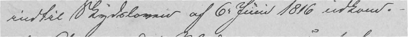indtil Skydsloven af 6. Juni 1816 udkom. -
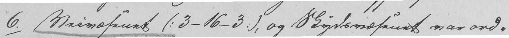6. Veivæsenet (: 3-16-3 :), og Skydsvæsenet var ord-
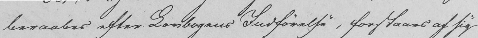beraabes efter Lovbogens Indførelse, forstaaes af sig
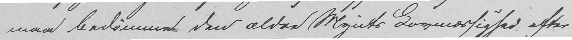maa bedømme den ældre Mynts Lovmæssighed efter
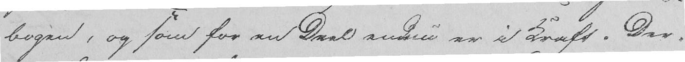bogen, og som for en Deel endnu er i Kraft. Der-
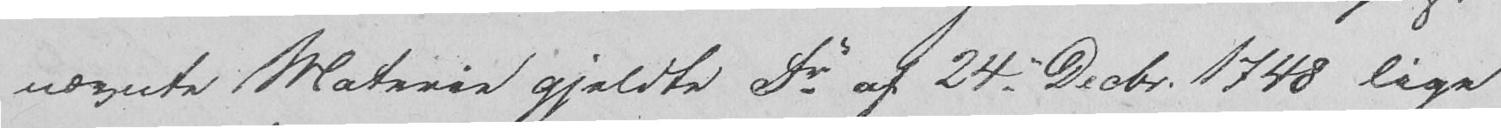nævnte Materie gjeldte Fr af 24. Decbr. 1748 lige
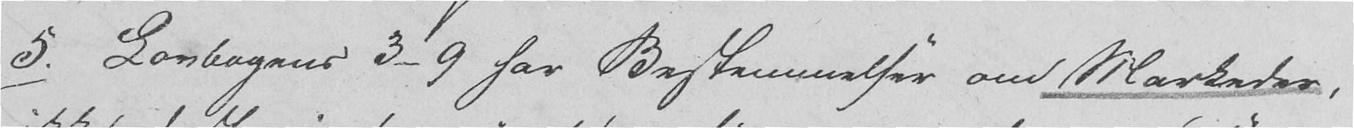5. Lovbogens 3-9 har Bestemmelser om Markeder,
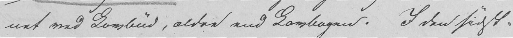net ved Lovbud, ældre end Lovbogen. I den sidst-
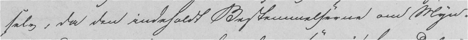selv, da den indeholdt Bestemmelserne om Myn-
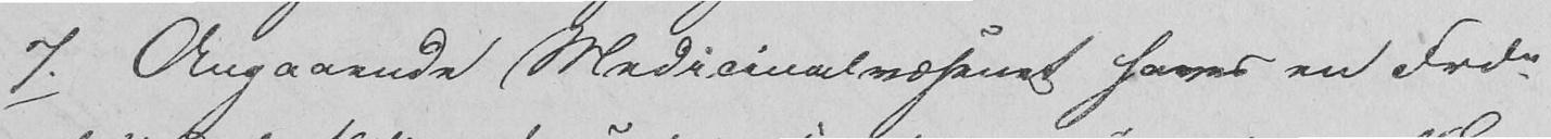7. Angaaende Medicinalvæsenet haves en Frdn
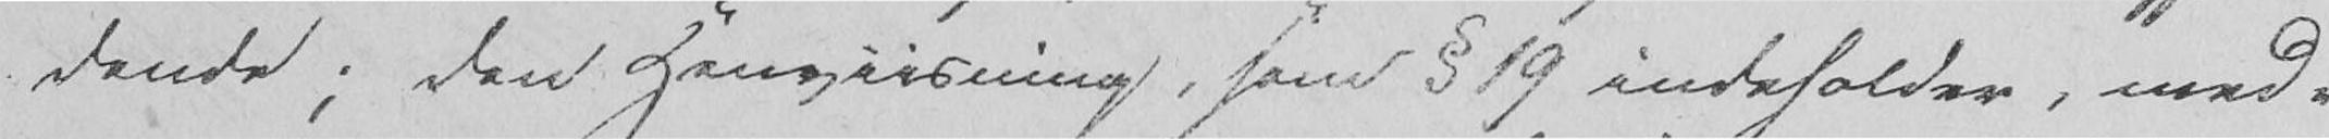dende; den Henviisning, som § 19 indeholder, med-
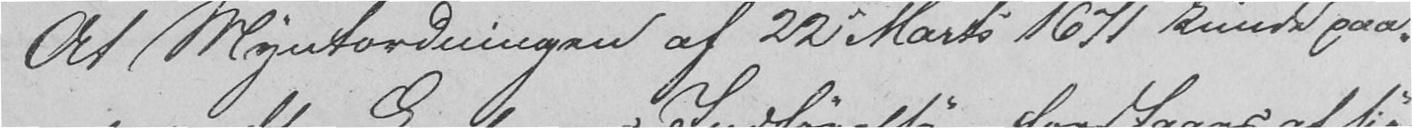At Myntordningen af 22 Marts 1671 kunde paa-
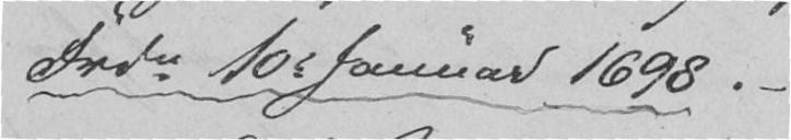Frdn 16. Januar 1698. -
